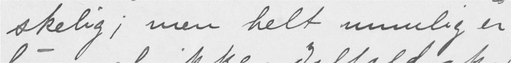skelig, men helt umulig er
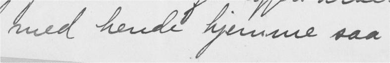med hende hjemme saa
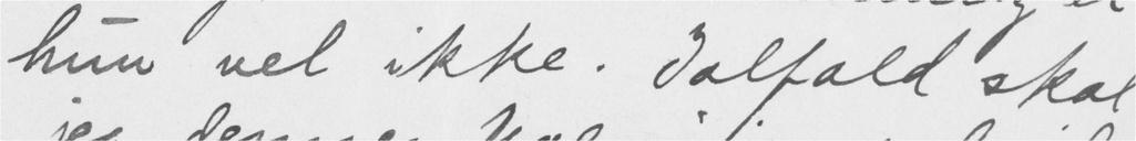hun vel ikke. Ialfald skal
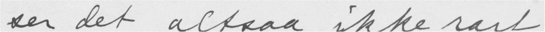ser det altsaa ikke rart
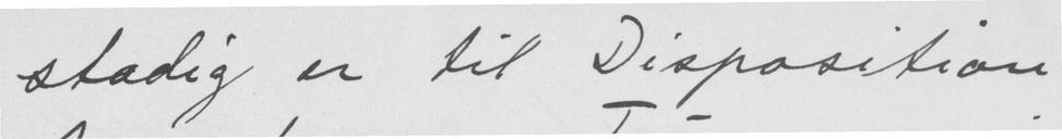stadig er til Disposition
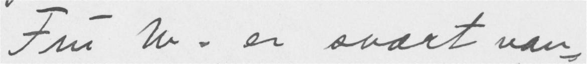Fru W. er svært van-
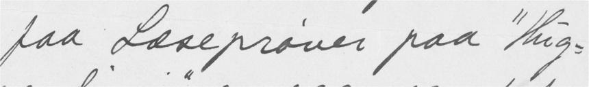faa Læseprøver paa "Hug-
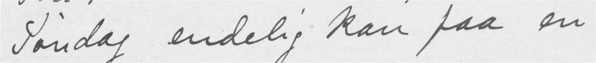Søndag endelig kan faa en
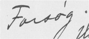Forsøg.
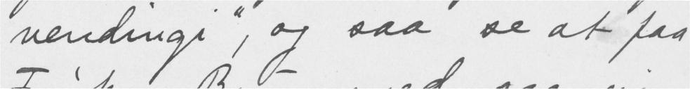vendingi", og saa se at faa
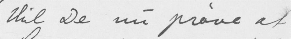Vil De nu prøve at
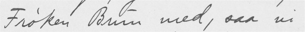Frøken Brun med, saa vi
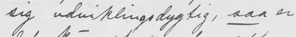sig udviklingsdygtig, saa er
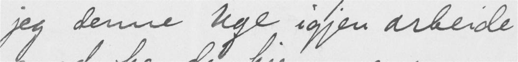jeg denne Uge igjen arbeide
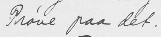Prøve paa det.
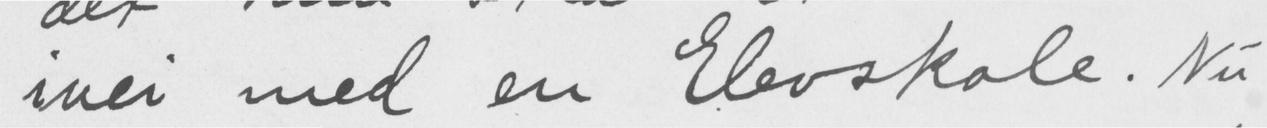ivei med en Elevskole. Nu
